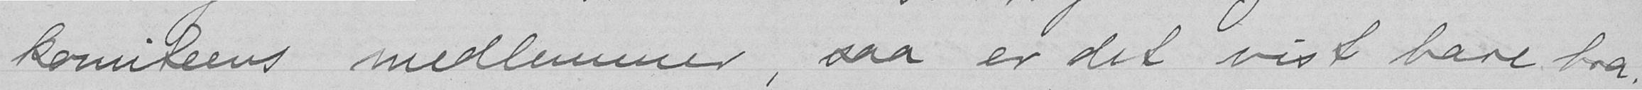komiteens medlemmer , saa er det vist bare bra.
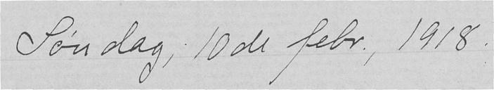Søndag, 10de febr., 1918.
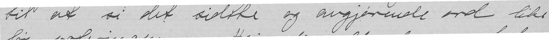til at si det sidste og avgjørende ord lile
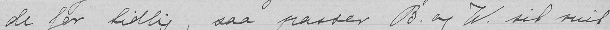de jer tidlig, saa paaser B. og W. sit snit
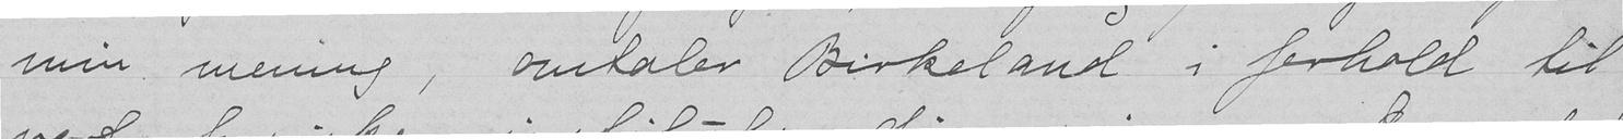min mening, omtaler Birkeland i forhold til
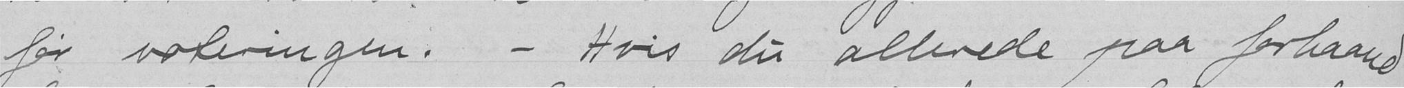før voteringen: - Hvis du allerede paa forhaand
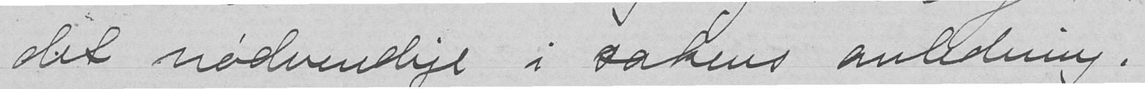det nødvendige i sakes anledning.
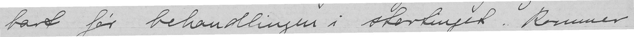bart før behandlingen i stortinget . Kommer
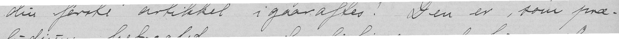den første artikkel igaaraftes! Den er, som præ-
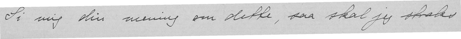Si mig din mening om dette, saa skal jeg straks
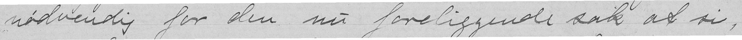nødvendig for den nu foreliggende sak at si,
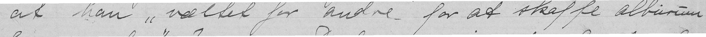at han "væltet for andre- for at skaffe alburum
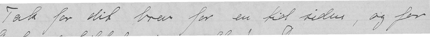Tal for dit brev for en tid siden, og for
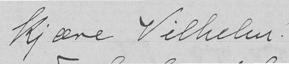Kjære Vilhelm.
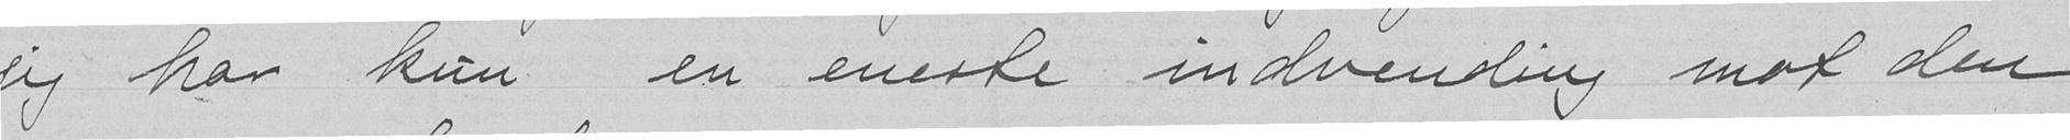jeg har kun en eneste indvending mod den
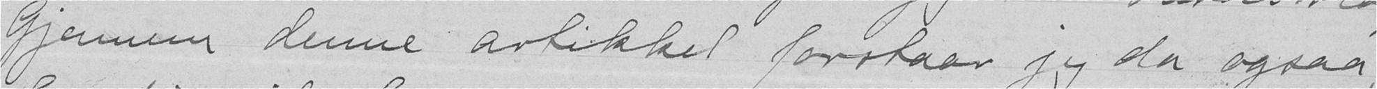Gjennem denne artikkel forstaar jeg da ogsaa
